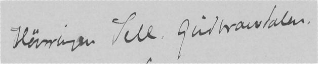Høvringen Tell, Gudbransdalen.
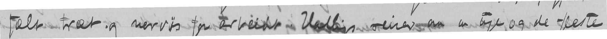fælt træt og nervøs for arbeidet. Hallings reiser om en uge og de fleste
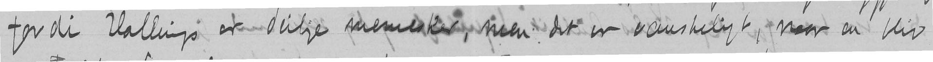fordi Hallings er deilige mennesker, men det er vanskeligt, naar en blir
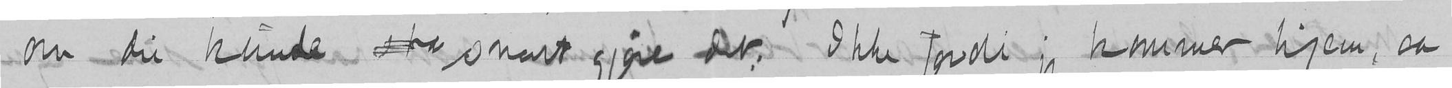om du kunde str snart gjøre det. Ikke fordi jeg kommer hjem, aa
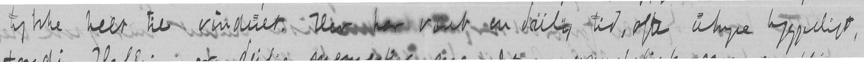tykke helt til vinduet. Her har været en deilig tid, ofte uhyre hyggeligt,
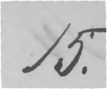15.
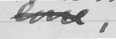lone,
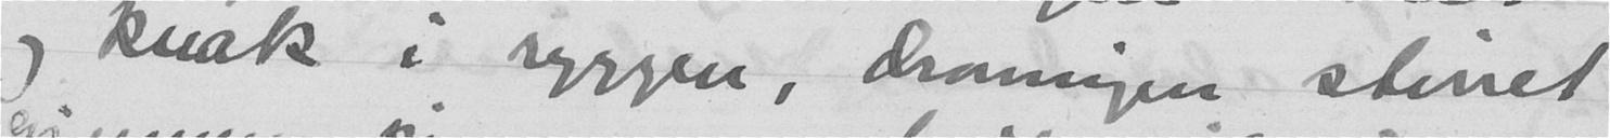og knak i ryggen, dronningen stirret
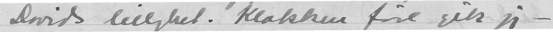Davids leilighet. Klokken fire gik jeg -
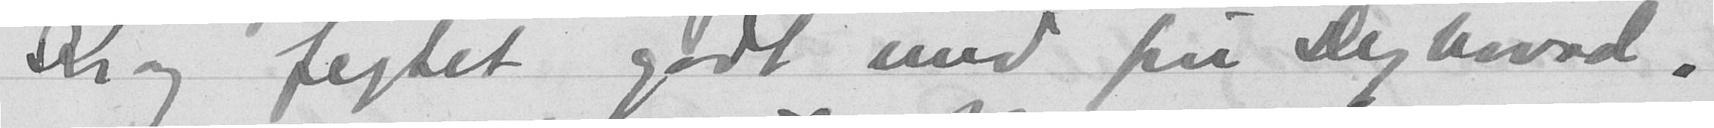Krog fegtet godt med fru Dybwad,
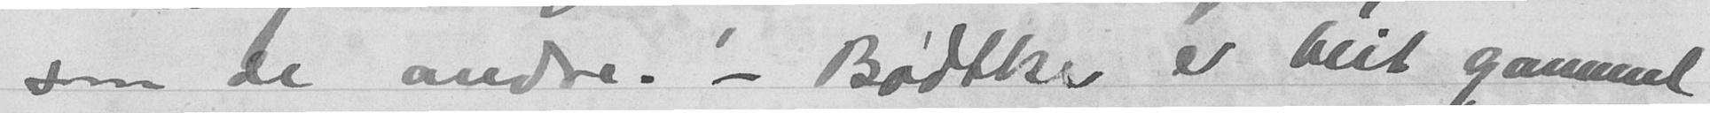som de andre. - Bødtker er blit gammel
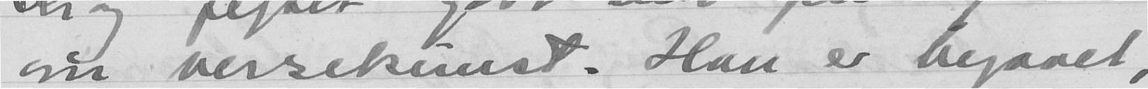om versekunst. Han er begavet,
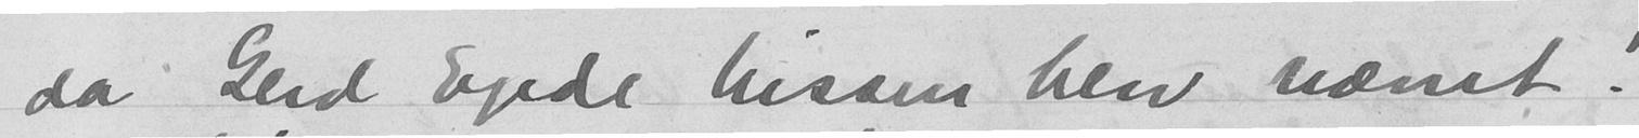da Gerd Egede Nissen blev nævnt!
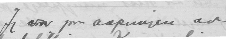Jeg var paa aapningen av
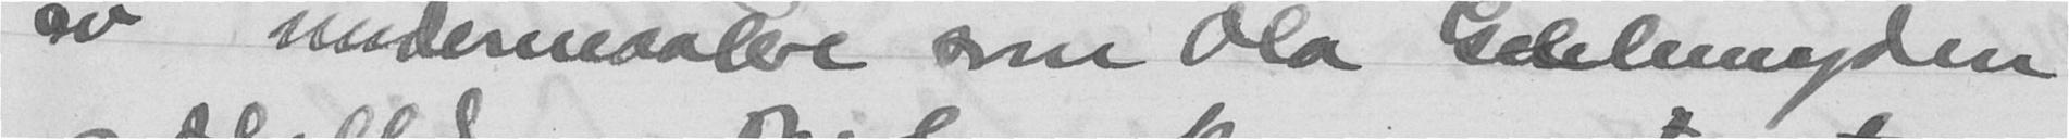av undermaalere som Ola Geelmuyden
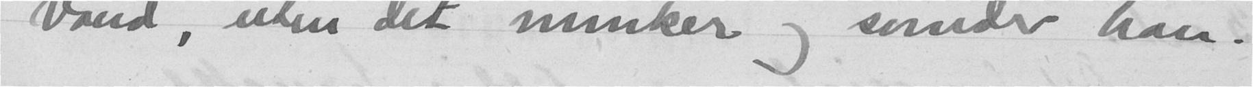vand, uten det minker og svinder han.
